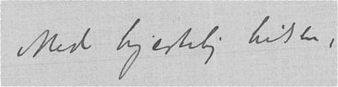Med hjertelig hilsen,
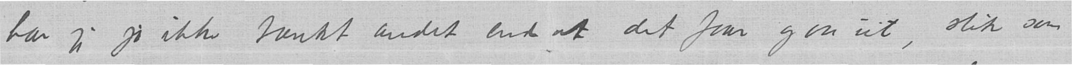har jeg jo ikke tænkt andet end at det faar gaa ut, slik som
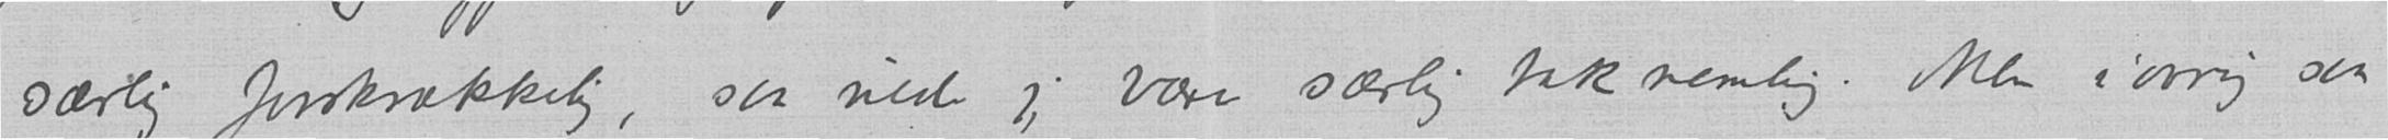særlig forskrækkelig, saa vilde jeg være særlig taknemlig. Men iøvrig saa
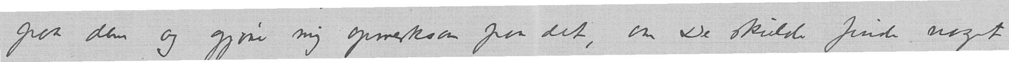paa dem og gjøre mig opmerksom paa det, om De skulde finde noget
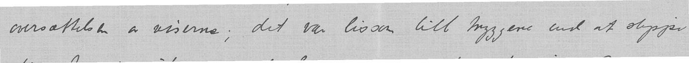oversættelsen av viserne; det var lissom litt tryggere end at slippe
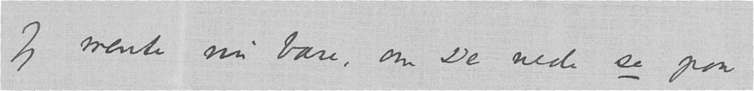Jeg mente nu bare, om De vilde se paa
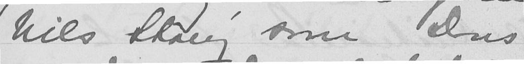Nils Stang som Dons
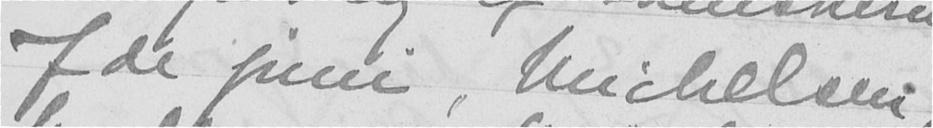7de juni, Michelsen,
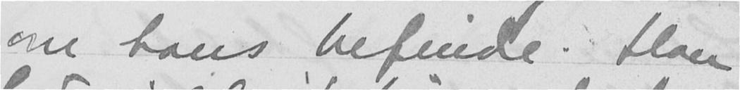om hans befinde. Han
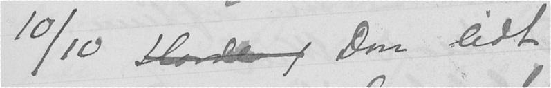10/10 Havde j Don lidt
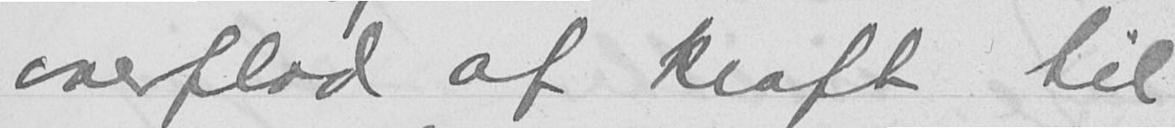overflod af kraft til
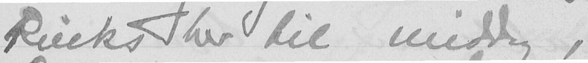Riecks her til middag,
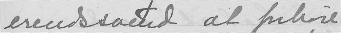erendsvend at forhøre
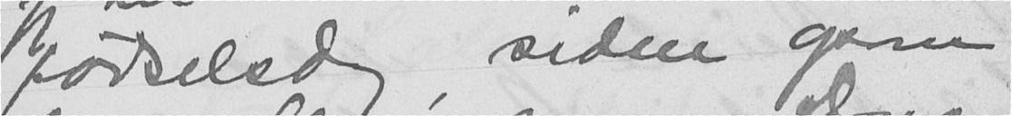fødselsdag, siden opom
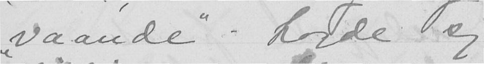"vaande". Lagde sig
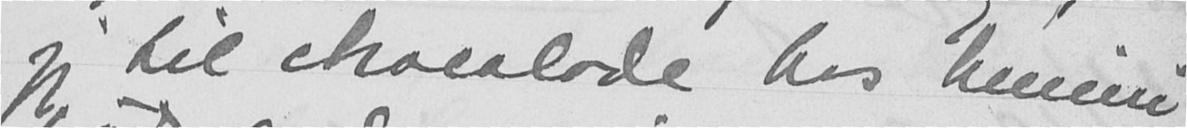jeg til chocolade hos Minni
